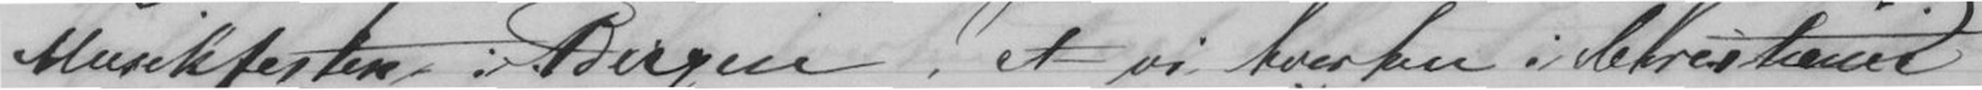Musikfesten i Bergen, at vi hverken i Christiania
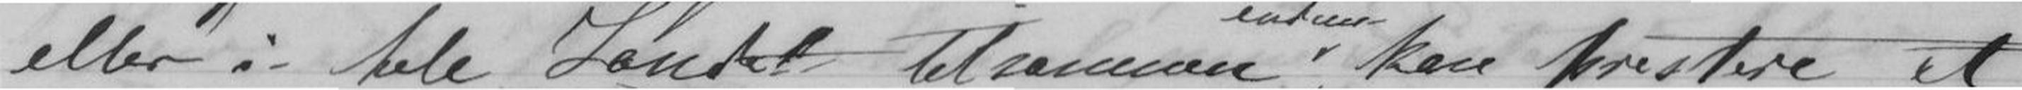eller i hele Landet tilsammen kan prestere et
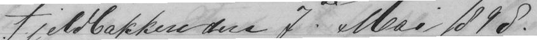Fjeldbakken den 7de Mai 1898.
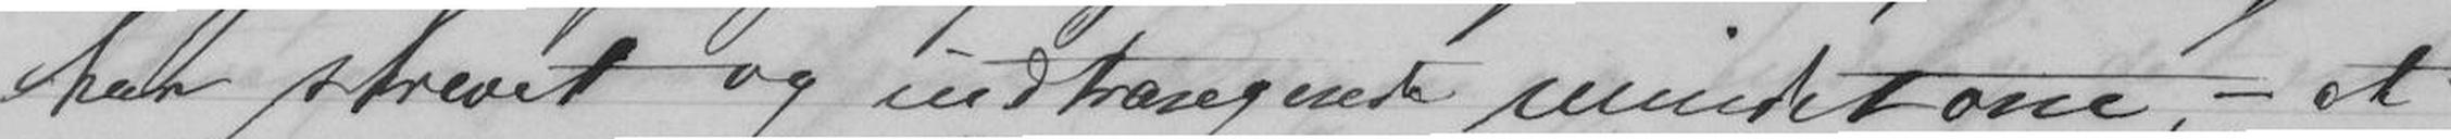har skrevet og indtrængende mindet om, - at
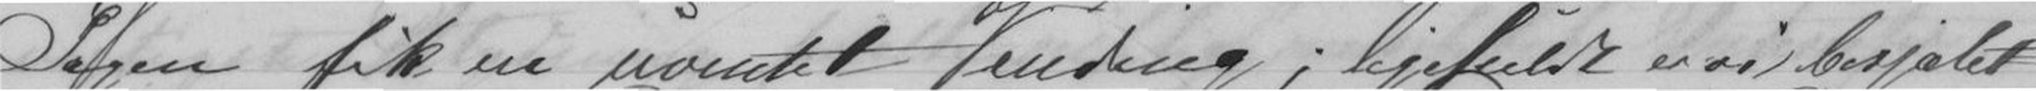Sagen fik en uventet Vending; ligefuldt er vi besjælet
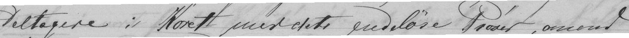Deltagere i Koret med dets endeløse Prøver, omend
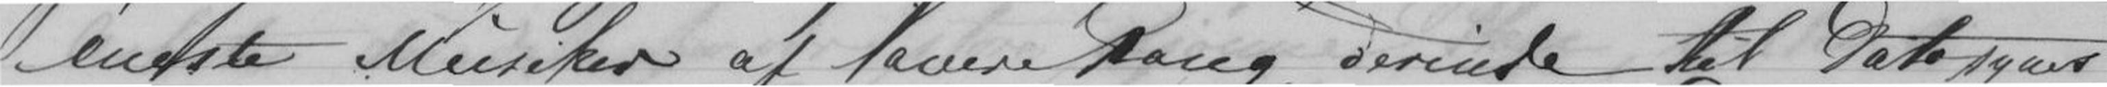éneste Musiker af lavere Rang derinde til Dato synes
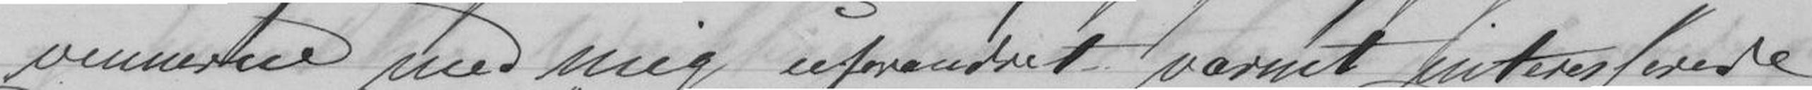vennerne med mig uforandret varmt interesserede
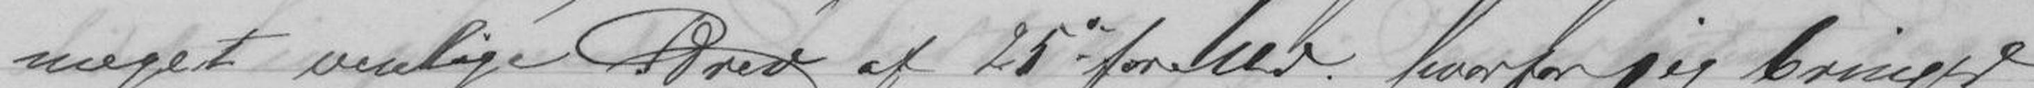meget venlige Brev af 25de forMd. hvorfor jeg bringer
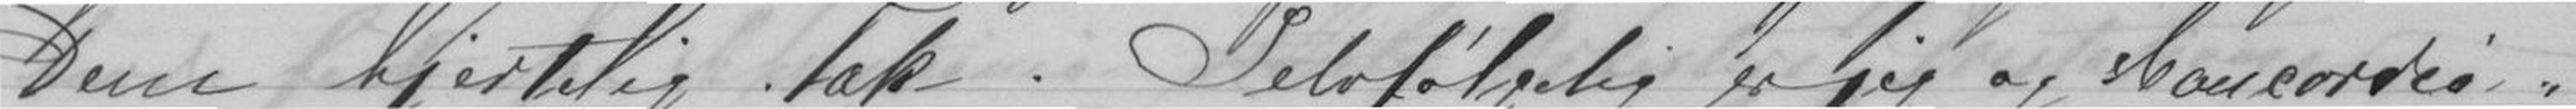Dem hjertelig Tak. Selvfølgelig er jeg og Concordie
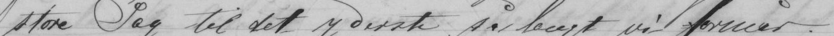store Sag til det yderste så langt vi formår.
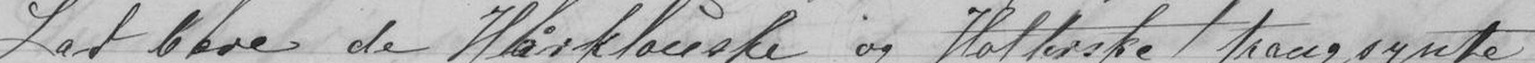Lad bare de Hårklouske og Holterske trangsynte
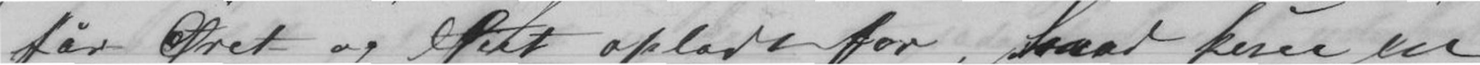får Øret og Øiet opladet for, hvad kun én
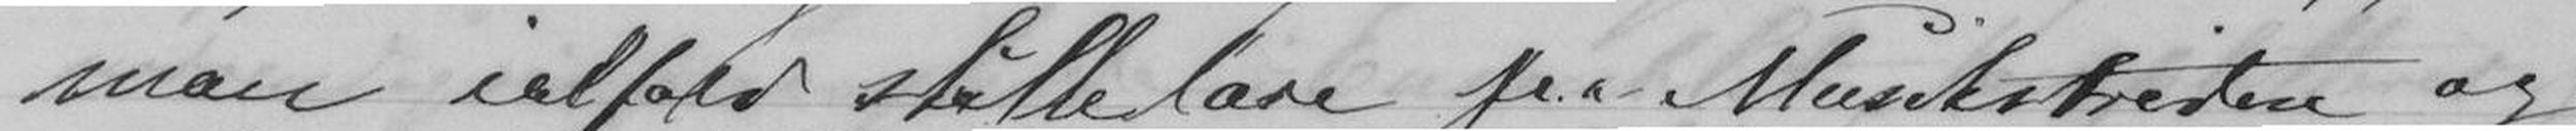man ialfald skulde lærs fra Musikstriden og
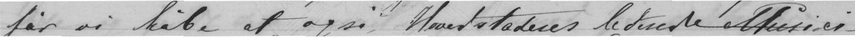får vi håbe, at også Hovedstadens ledende Musici
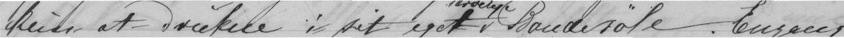kun at drukne i sit eget Bandesøle. Engang
